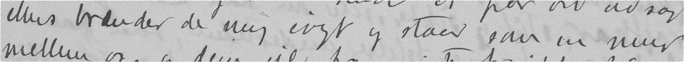ellers brænder de mig evigt og staar som en mur
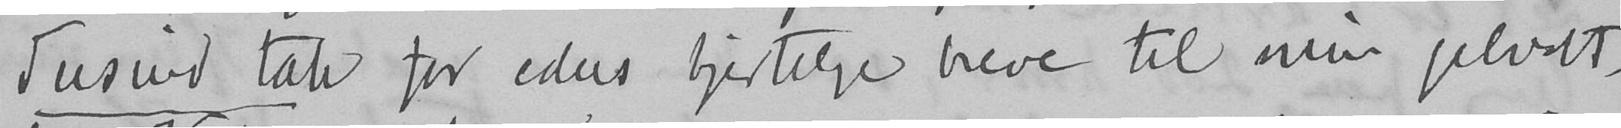Tusind tak for eders hjertelige breve til min geburst-
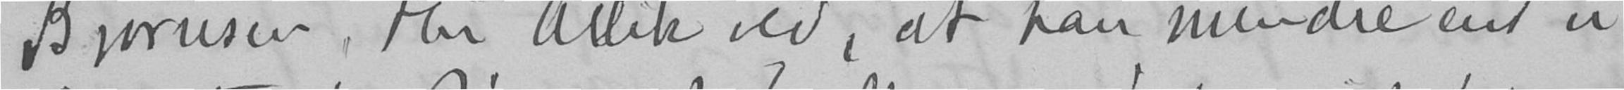Bjørnson, thi Allik ved, at han mindre end vi
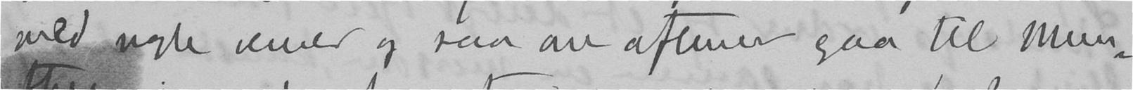med nogle venner og saa om aftenen gaa til Mun-
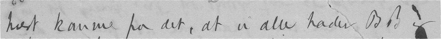hvert komme fra det, at vi alle hader BB og
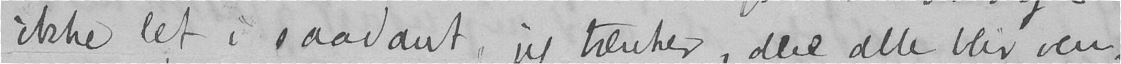ikke let i saadant, jeg tænker, dere alle blir ven-
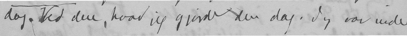dag. Ved dere, hvad jeg gjorde den dag. Jeg var inde
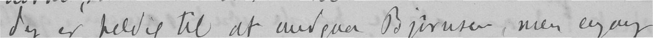Jeg er heldig til at undgaa Bjørnson, men engang
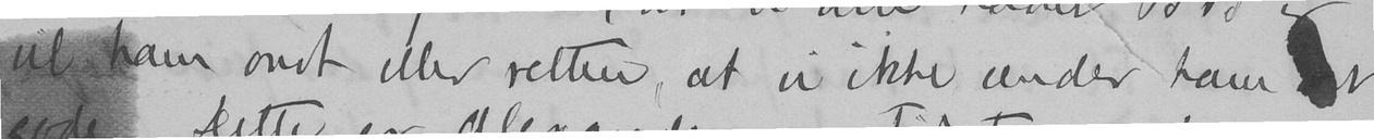vil ham ondt eller rettere, at vi ikke under ham det
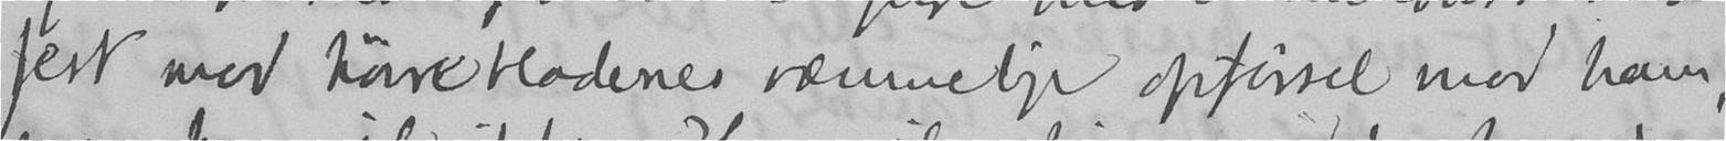fest mod høirebladenes væmmelige opførsel mod ham,
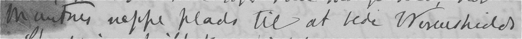Munthes neppe plads til at bede Werenskiolds
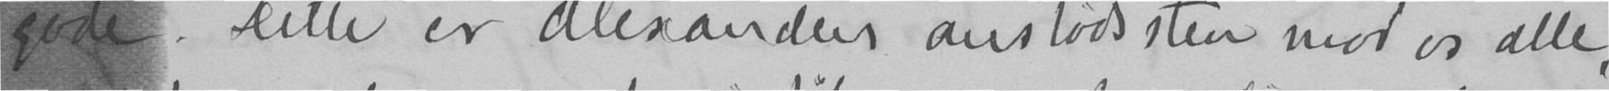gode. Dette er Alexanders anstødssten mod os alle,
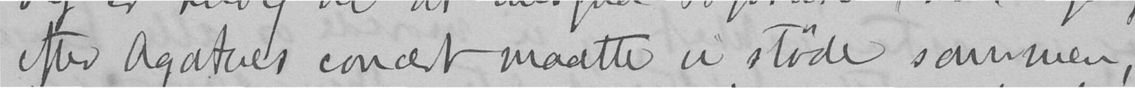efter Agathes concert maatte vi støde sammen,
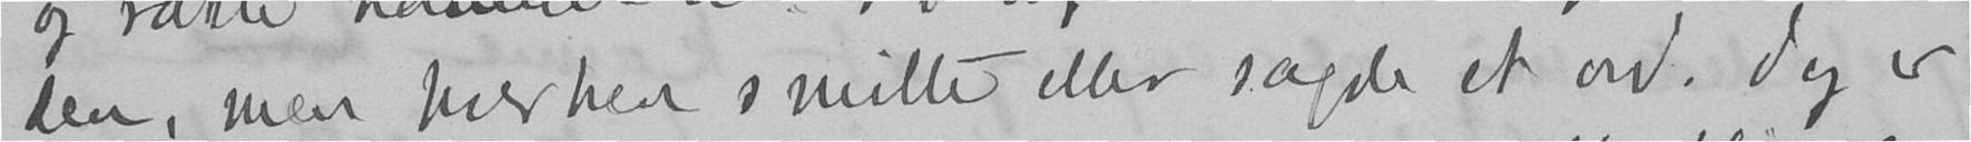den, men hverken smilte eller sagde et ord. Jeg er
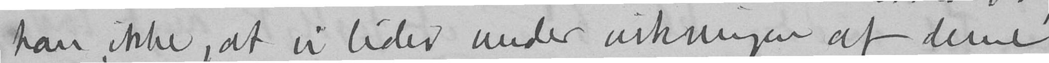han ikke, at vi lider under virkningen af denne
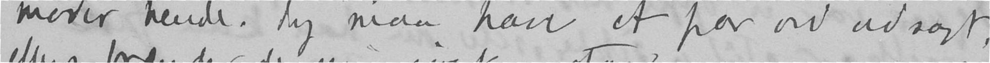møder hende. Jeg maa have et par ord udsagt,
